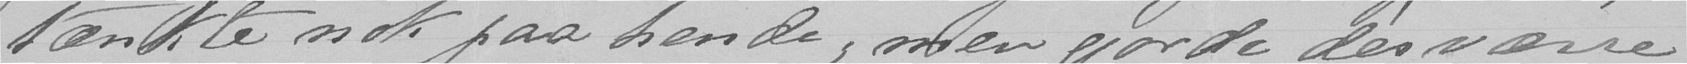tænkte nok paa hende, men gjorde desværre
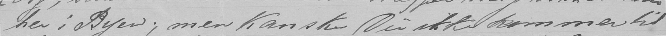her i Byen; men kanske Du ikke kommer til
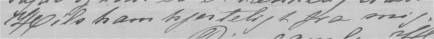Hils ham hjerteligt fra mig.
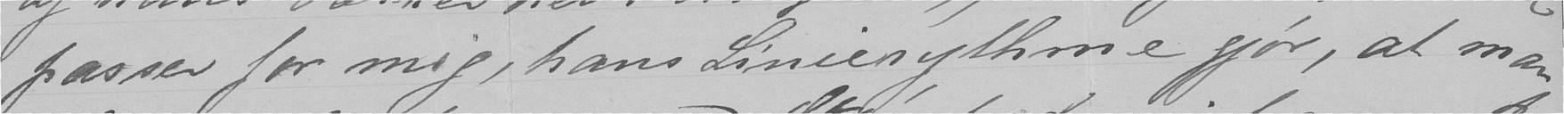passer for mig, hans Linierythme gjør, at man
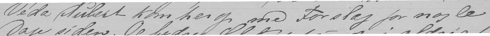Véda Aubert kom herop med Forslag for nogle
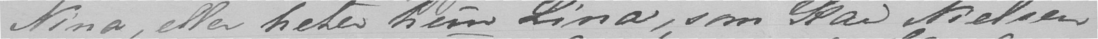Nina, eller heter hun Lina, som Kai Nielsen
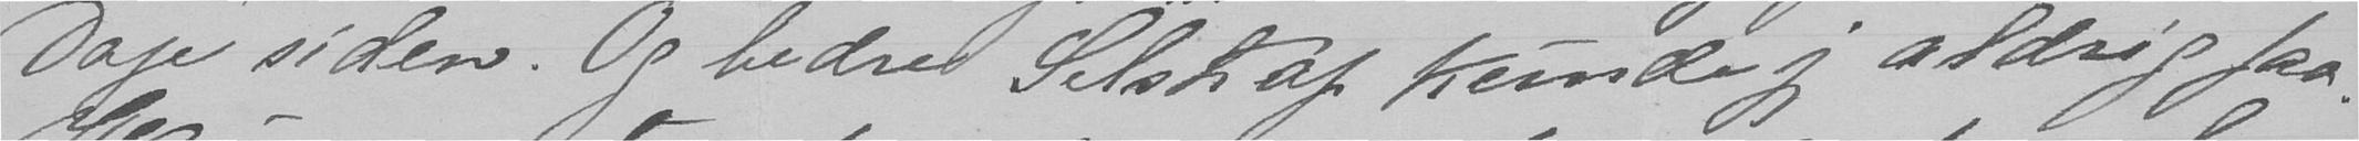Dage siden. Og bedre Selskap kunde jeg aldrig faa.
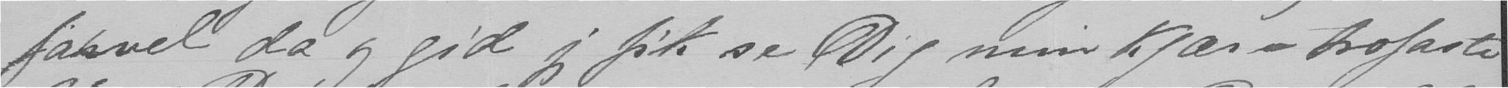farvel da og gid jeg fik se Dig min kjære trofaste
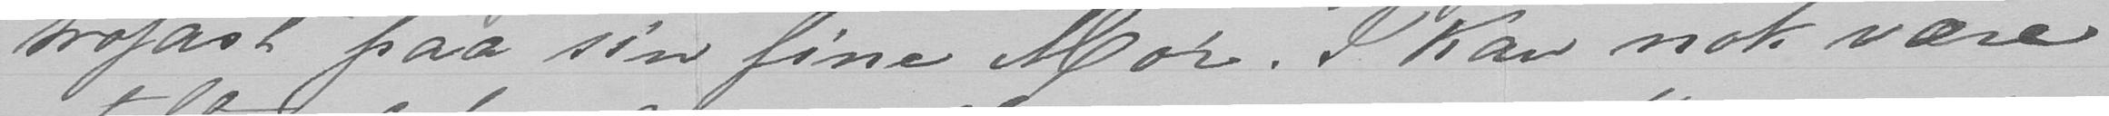trofast paa sin fine Mor. I kan nok være
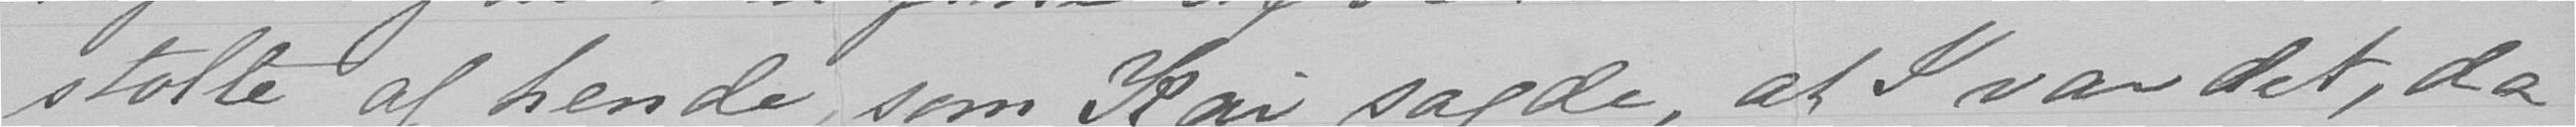stolte af hende, som Kai sagde, at I var det, da
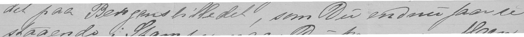det paa Bergensbilledet, som Du endnu faar se
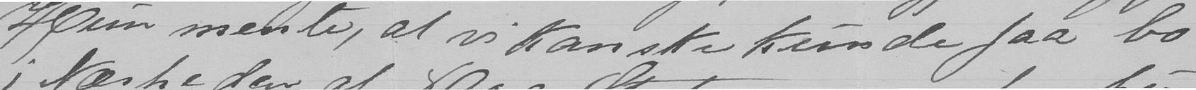Hun mente, at vi kanske kunde faa bo
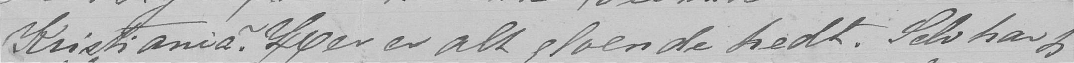Kristiania. Her er alt gloende hedt. Selv har jeg
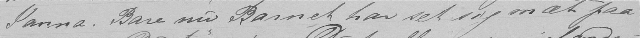Janna. Bare nu Barnet har set sig mæt paa
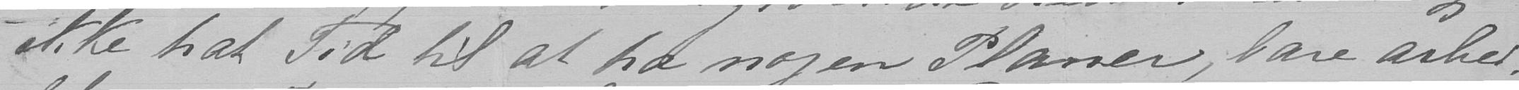ikke hat Tid til at ha nogen Planer, bare arbei-
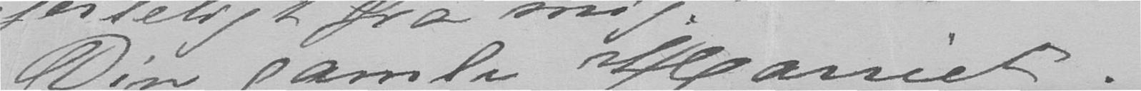Din gamle Harriet.
